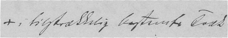i tilstrækkelig bestemte Træk
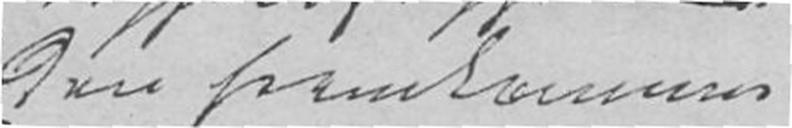den fremkommer
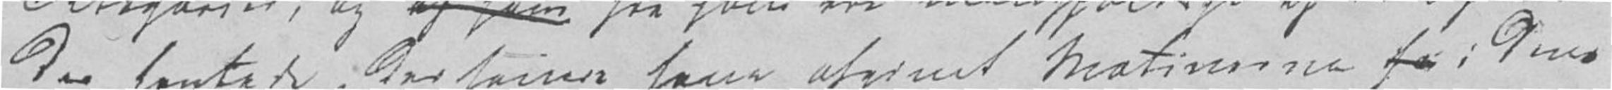der hentede, der senere have afgivet Motiverne for i dens
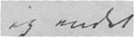og andet
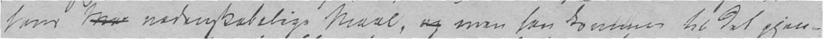hans Ma videnskabelige Maal, og men han kommer til det gjen-
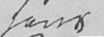hans
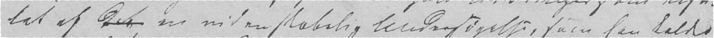tat af det en videnskabelig Undersøgelse, som han kalder
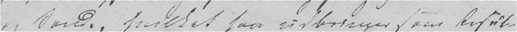og Sande, hvilket han udbringer som Resul-
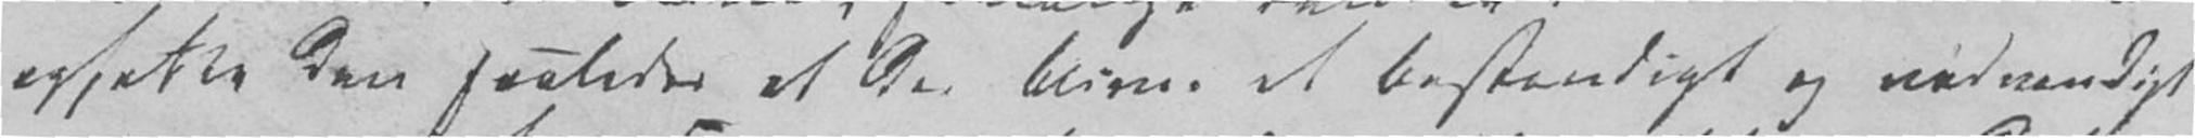opfatte den saaledes at der bliver et bestandigt og nødvendigt
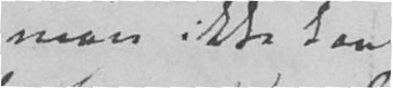man ikke kan
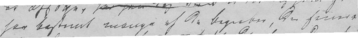har bestemt mange af de Begreber, der senere
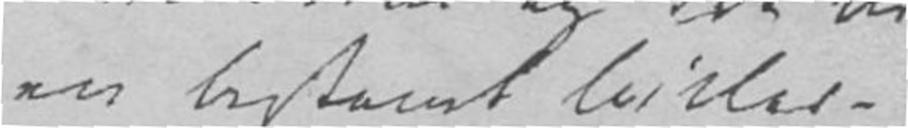en bestemt Billed-
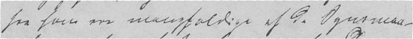fra ham ere mangfoldige af de Synsmaa-
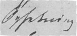Opfatning
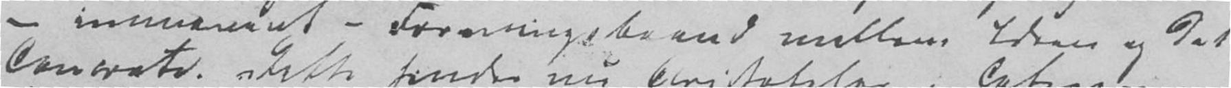- immanent - Foreningsbaand mellem Ideen og det
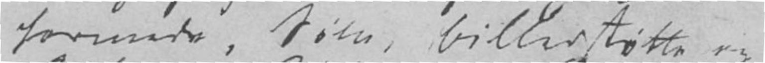formede, Sølv, Billedstøtte og
